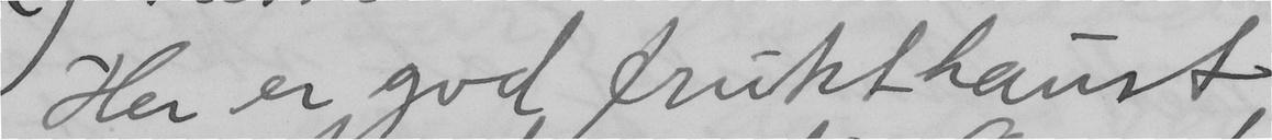Her er god frukthaust
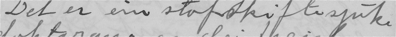Det er ein stofskiftesjuke
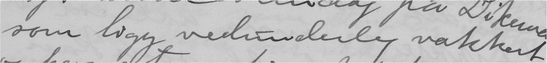som ligg vedunderleg vakkert
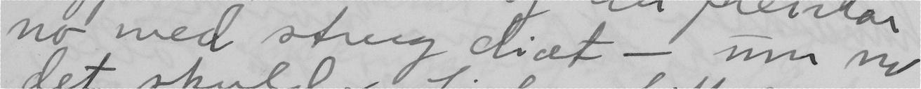no med streng diæt – um no
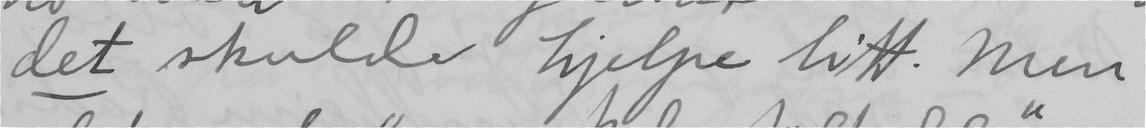det skulde hjelpe litt. Men
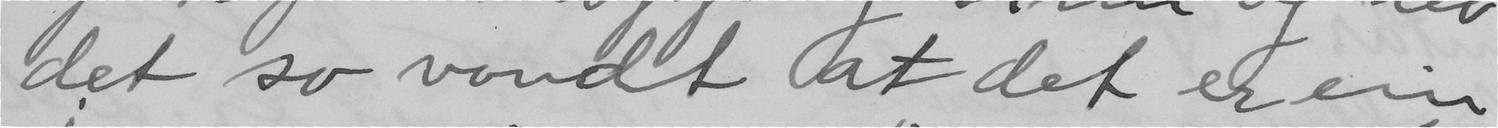det so vondt at det er ein
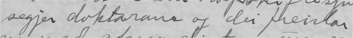segjer doktarane og dei freistar
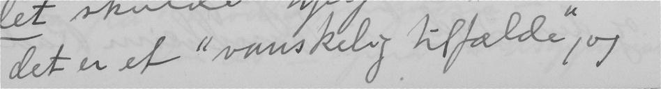det er et "vanskelig tilfælde", og
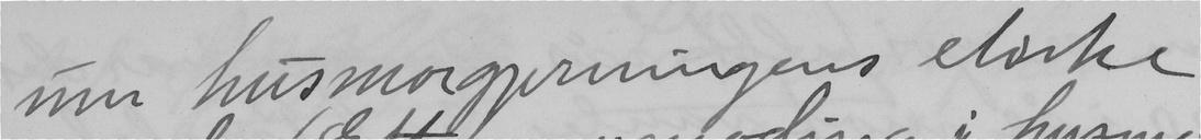um husmorgjerningens elorke
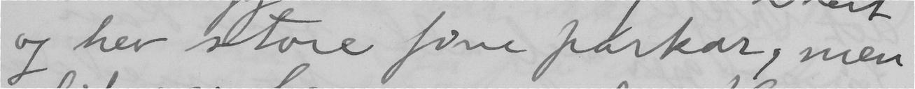og hev store fine parkar, men
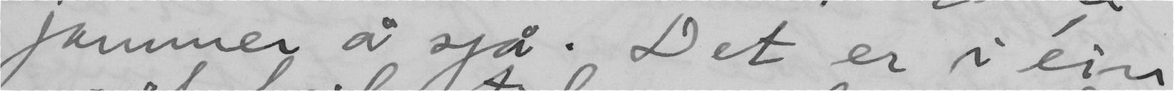jammer å sjå. Det er i ein
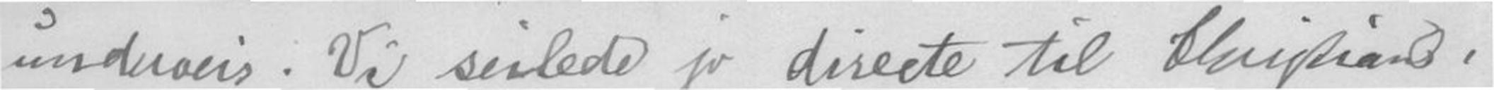underveis. Vi seilede jo directe til Christian-
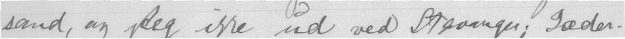sand, og steg ikke ud ved Stavanger; Jæder-
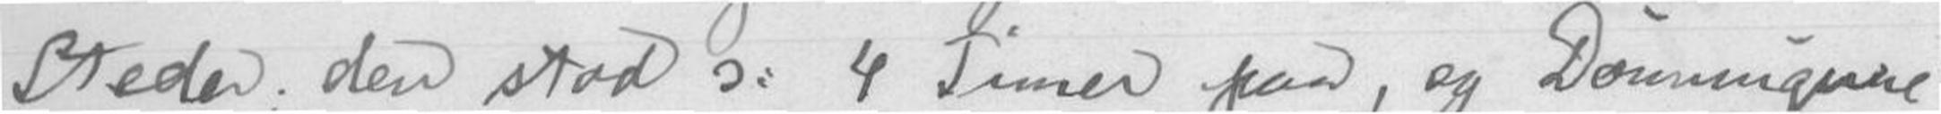Steder; den stod ): 4 Timer paa, og Dønningene
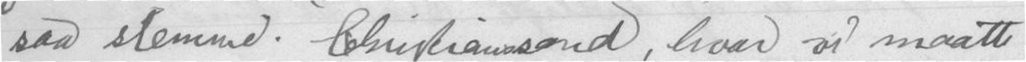saa slemme. Christiansand, hvor vi maatte
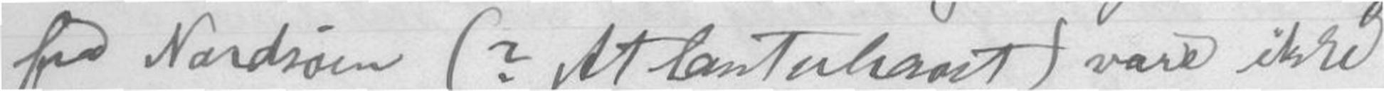fra Nordsøen (?Atlanterhavet) vare ikke
